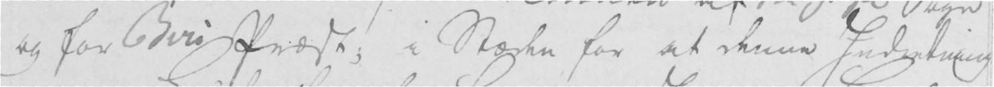og for Biri Præst; i Stæden for at denne Indretning
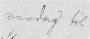verdag til
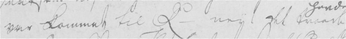var kommet til R - ney det troede
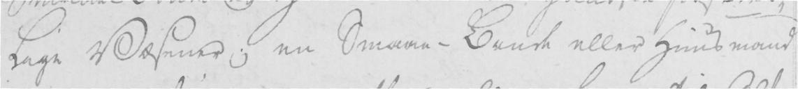lige Væsener; en Smaae-Bonde eller Huusmand
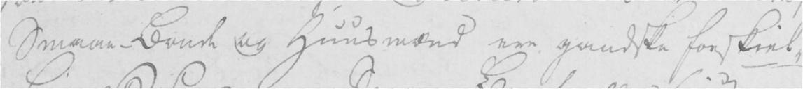Smaae-Bonde og Huusmænd er gandske forskiel-
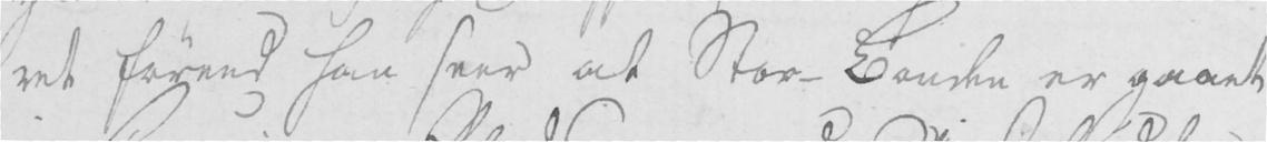ret førend han seer at Stor-Bonden er gaaet
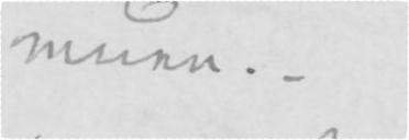muen. -
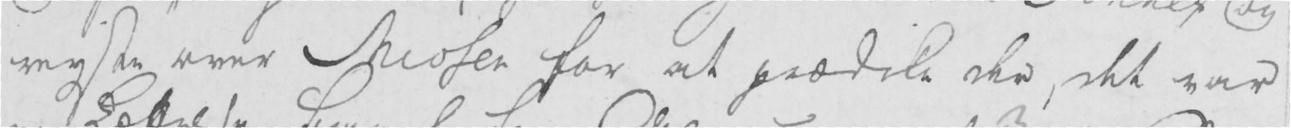reyste over Miøsen for at prædike der, det var
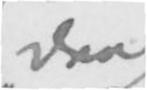den
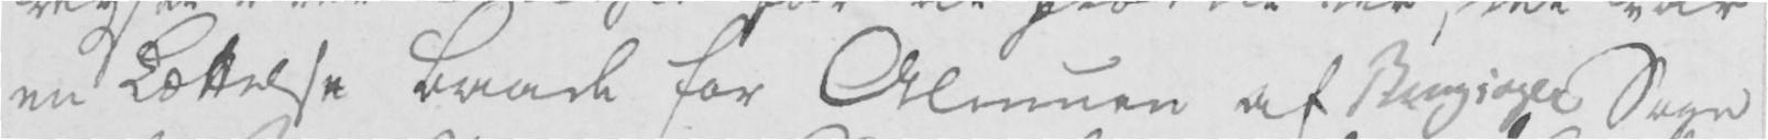en Lættelse baade for Almuen af Ringsager Sogn
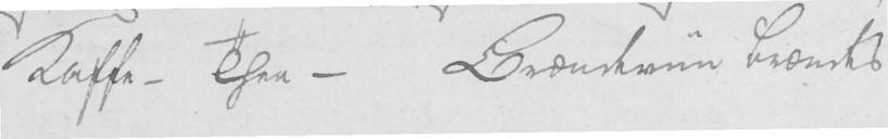Kaffe - Thee - Brændeviin brændes
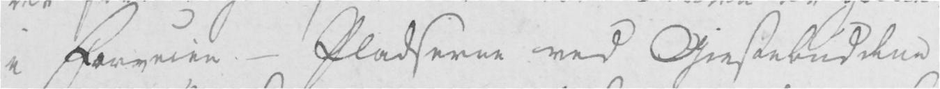i Forveien - Pladserne ved Giestebuddene
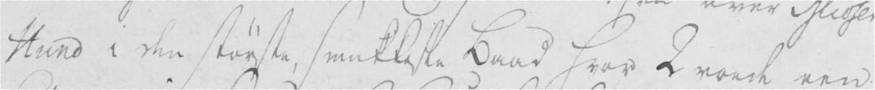stund i den største smukkeste Baad hvor 2 roede een
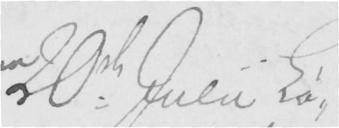20de Julii Lø-
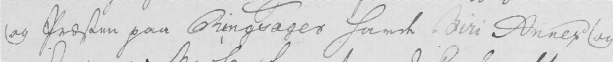og Præsten paa Ringsager havde Biri Annex og
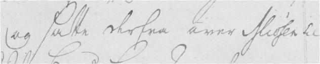og satte derfra over Miøsen en
